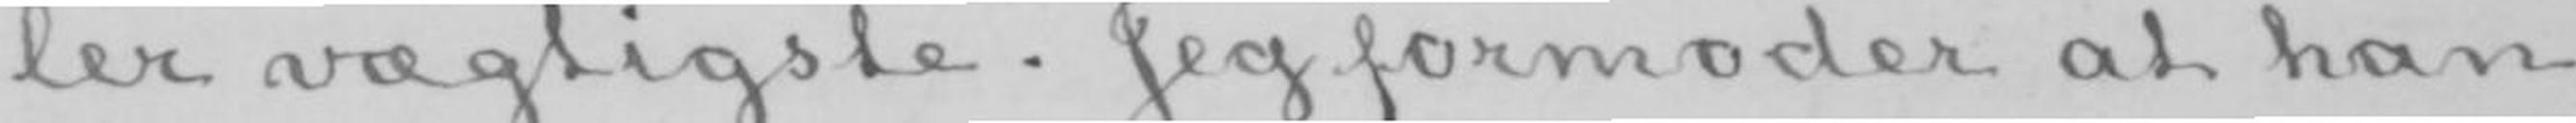ler vægtigste. Jeg formoder at han
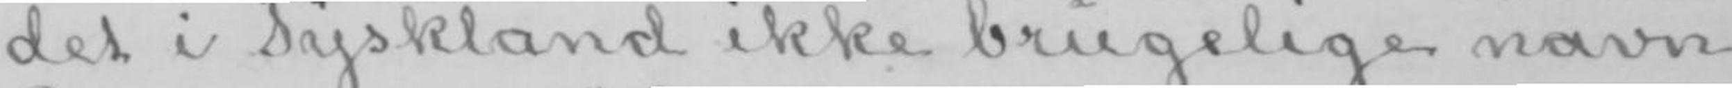det i Tyskland ikke brugelige navn
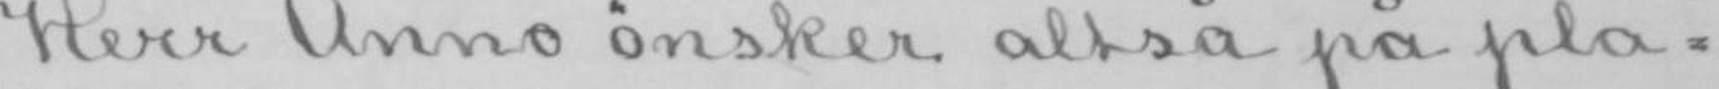Herr Anno ønsker altså på pla-
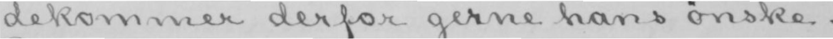dekommer derfor gerne hans ønske.
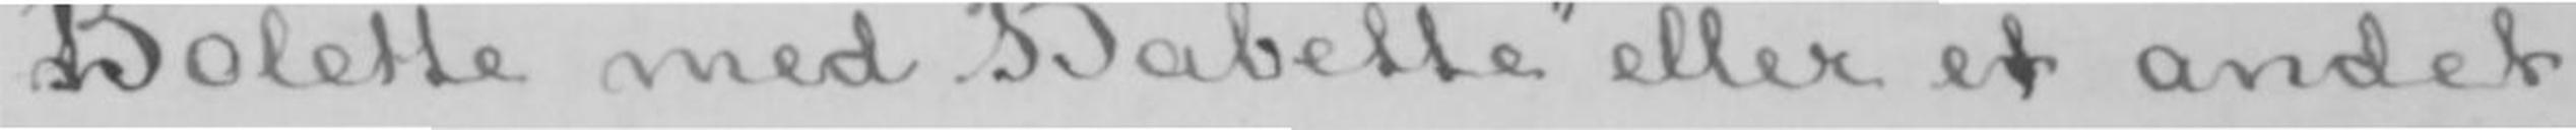Bolette med «Babette» eller et andet
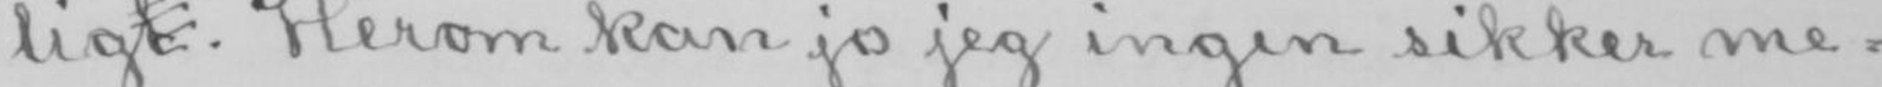ligt. Herom kan jo jeg ingen sikker me-
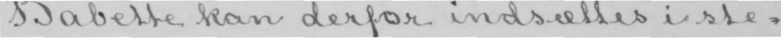Babette kan derfor indsættes i ste-
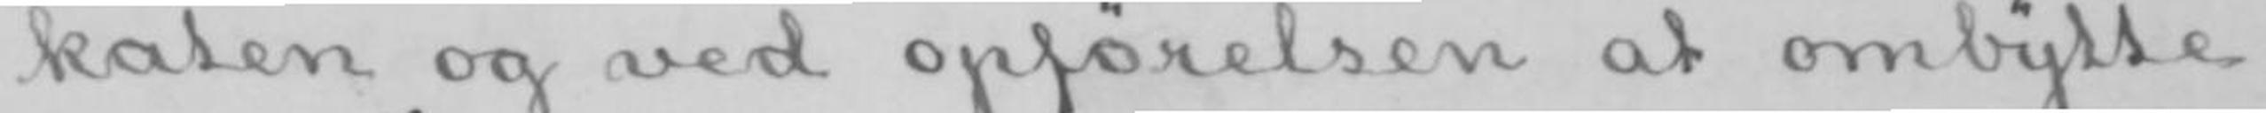katen og ved opførelsen at ombytte
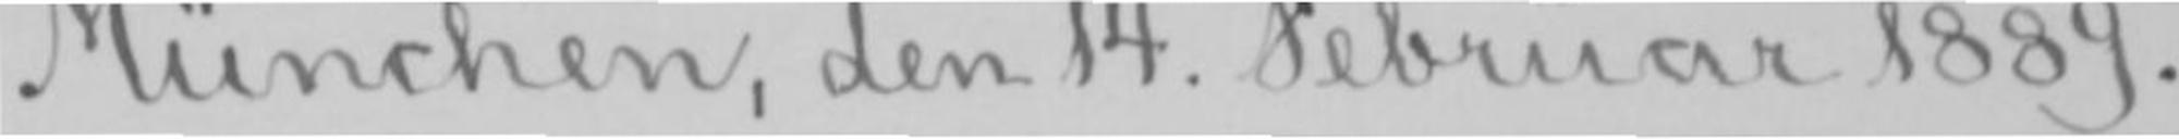München, den 14. Februar 1889.
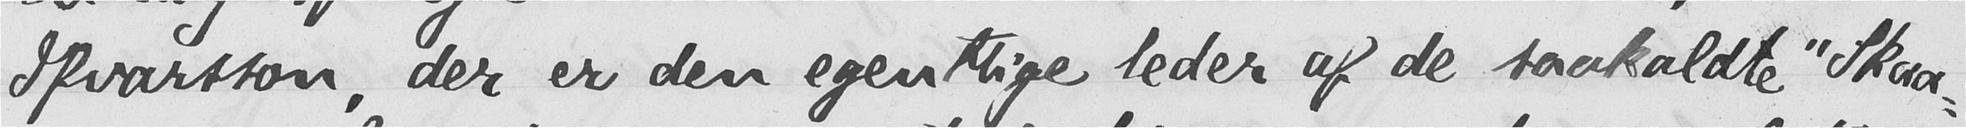Ifvarsson, der er den egentlige leder af de saakaldte "Skaa-
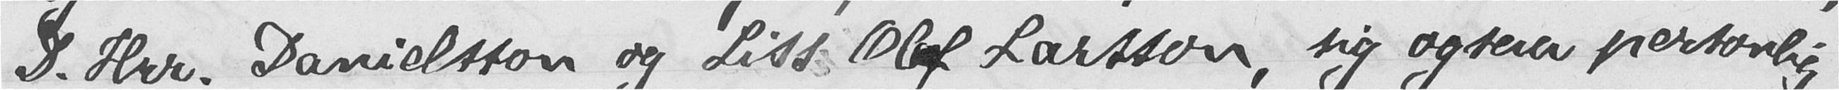D. Hrr. Danielsson og Liss Olof Larsson, sig ogsaa personlig
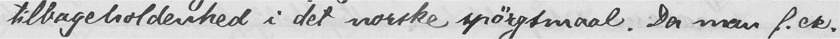tilbageholdenhed i det norske spørgsmaal. Da man f. ex.
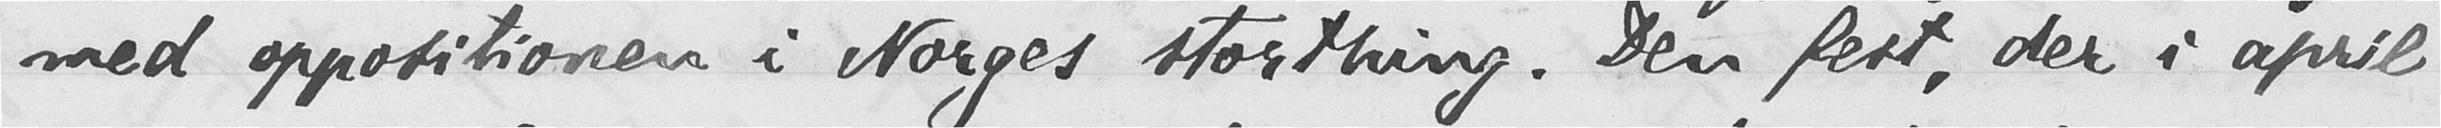med oppositionen i Norges storthing. Den fest, der i april
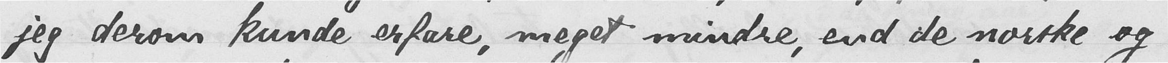jeg derom kunde erfare, meget mindre, end de norske og
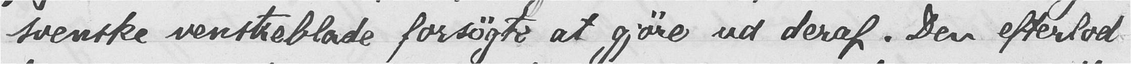svenske venstreblade forsøgte at gjøre ud deraf. Den efterlod
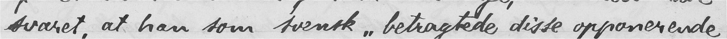svaret, at han som svensk "betragtede disse opponerende
